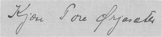Kjære Tore Ørjasæter
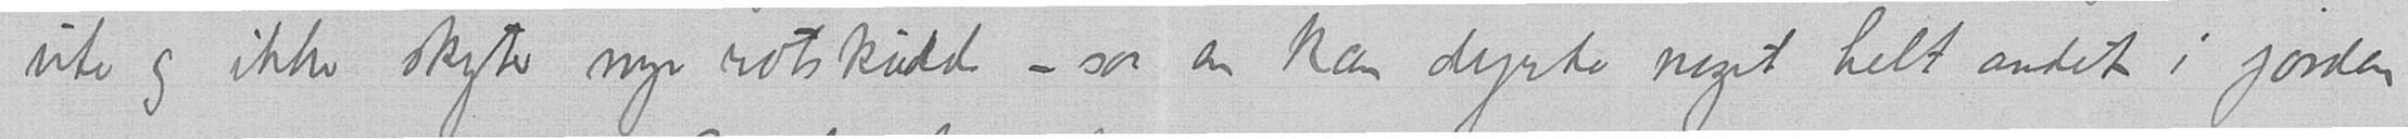ute og ikke skyter nye rotskudd – saa en kan dyrke noget helt andet i jorden
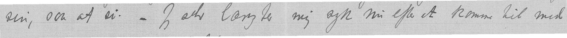sin, saa at si. – Jeg selv længter mig syk nu efter at komme til med
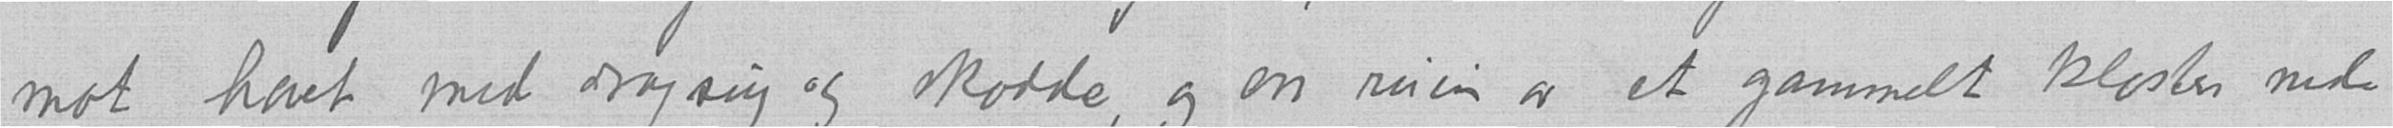mot havet med dragsug og skodde, og en ruin av et gammelt kloster nede
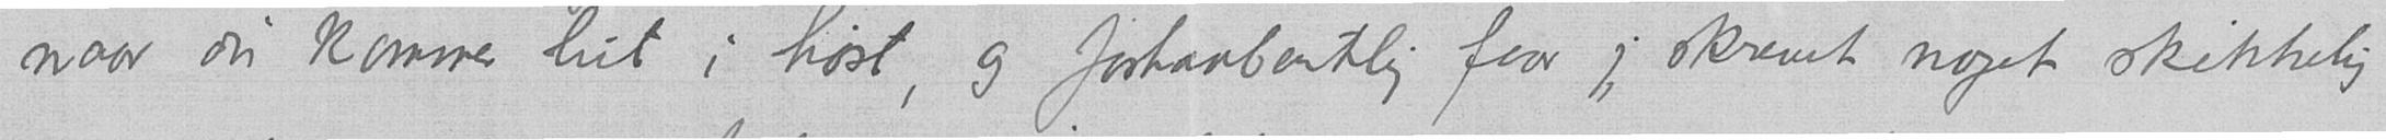naar du kommer hit i høst, og forhaabentlig faar jeg skrevet noget skikkelig
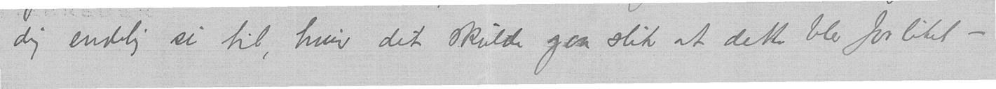dig endelig si til, hvis det skulde gaa slik at dette blev forlitet –
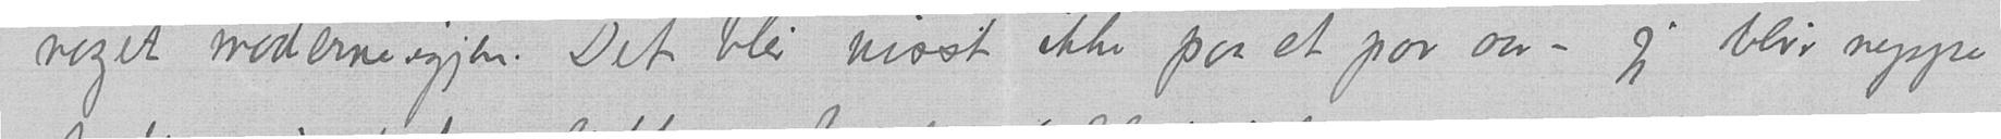noget moderne igjen. Det blir visst ikke paa et par aar – jeg blir neppe
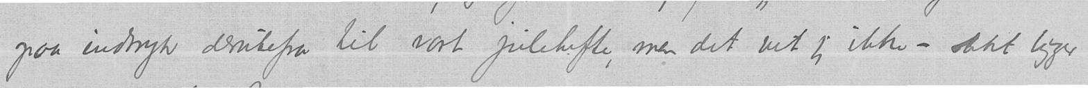paa indtryk derutefra til vort julehefte, men det vet jeg ikke – slikt ligger
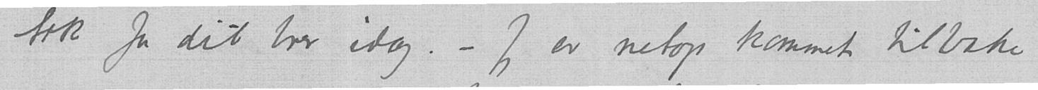tak for dit brev idag. – Jeg er netop kommet tilbake
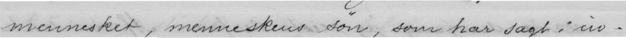mennesket, menneskens søn, som har sagt: in-
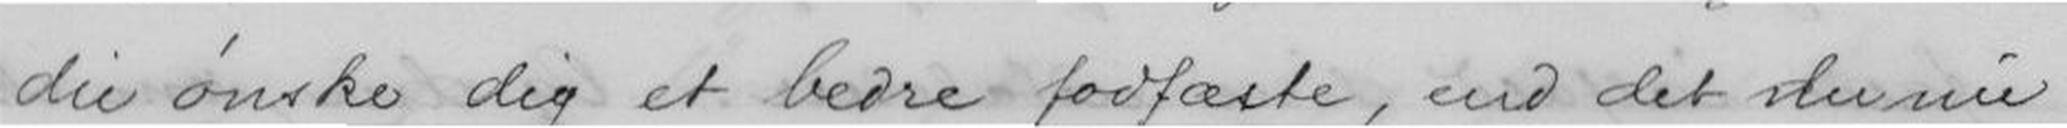du ønske dig et bedre fodfæste, end det du nu
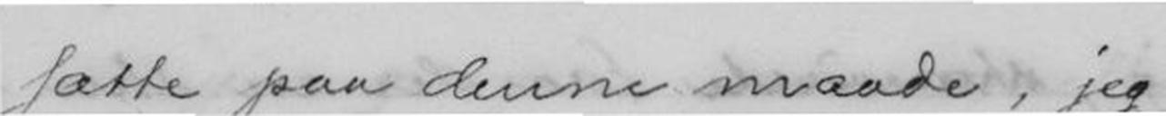sætte paa denne maade, jeg
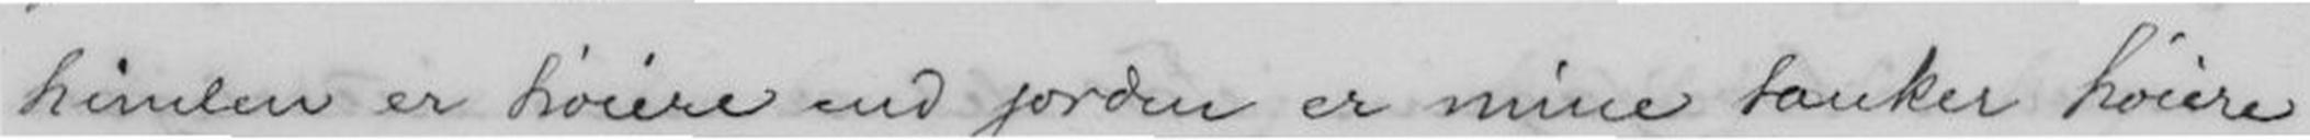himlen er høiere end jorden er mine tanker høiere
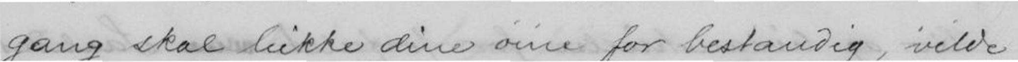gang skal lukke dine øine for bestandig, vilde
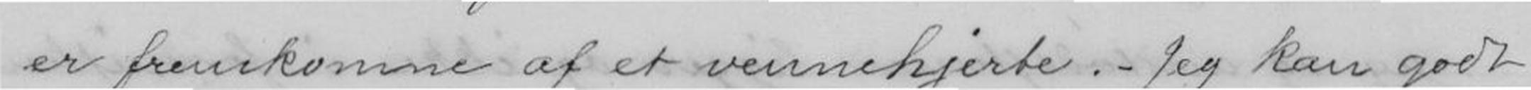er fremkomme af et vennehjerte. - Jeg kan godt
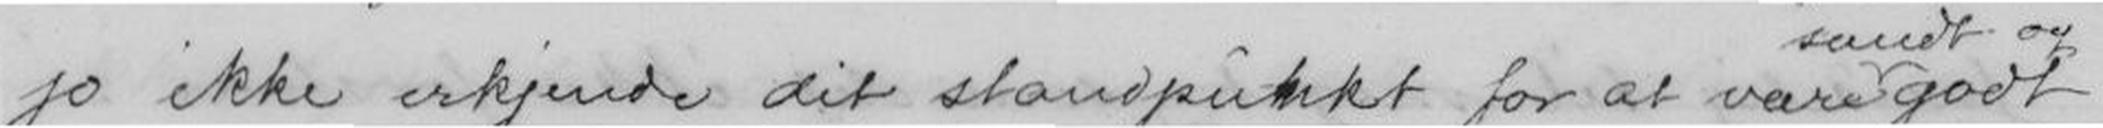jo ikke erkjende dit standpunkt for at være godt
